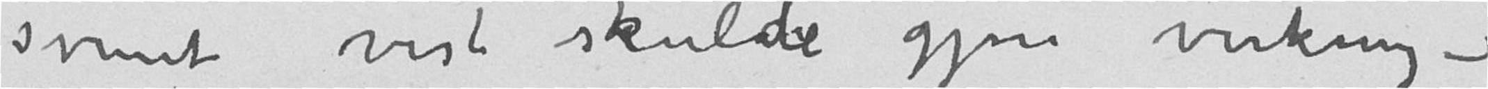svinet vist skulde gjøre virkning –
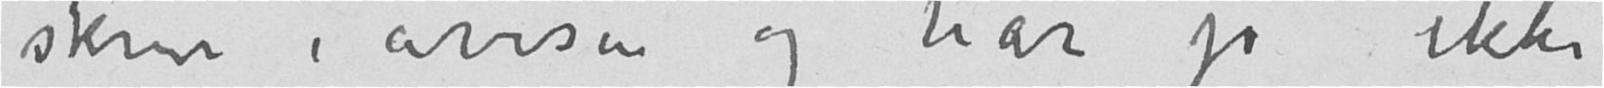skrive i avisen og har jo ikke
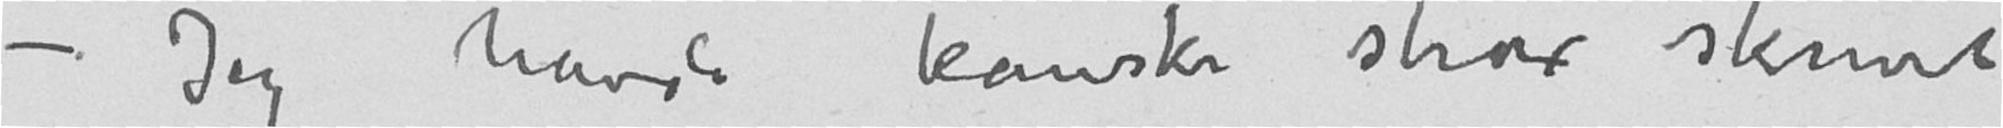– Jeg havde kanske strax skrevet
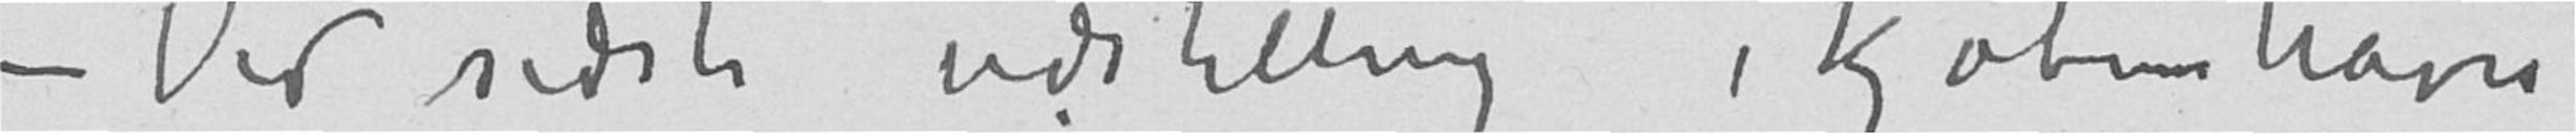– Ved sidste udstilling i Kjøbenhavn
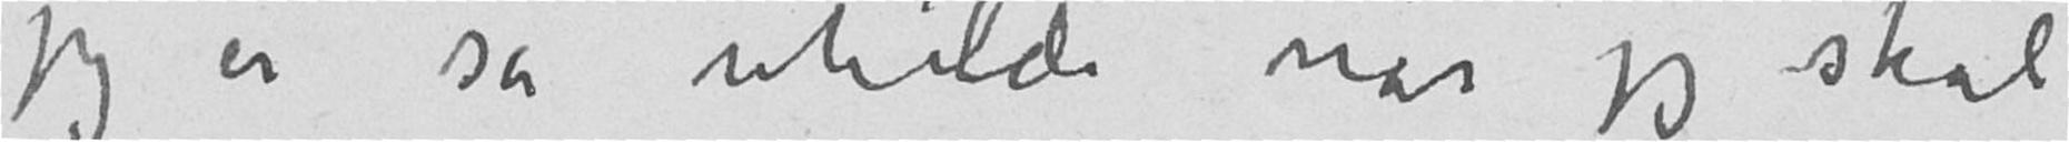jeg erså uheldi når jeg skal
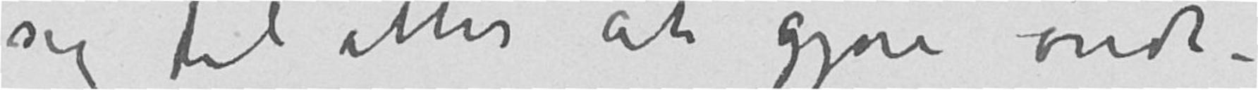sig til atter at gjøre ondt –
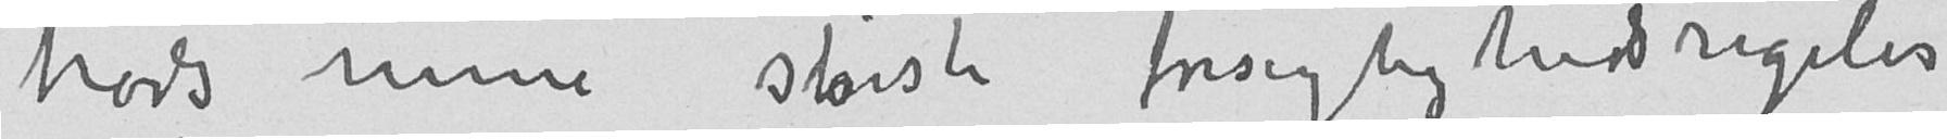tross mine stoiske forsigtighedsregeler
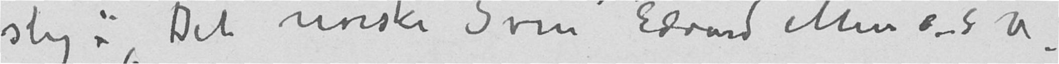slig: «Det norske Svin Edvard Munch o.s v.
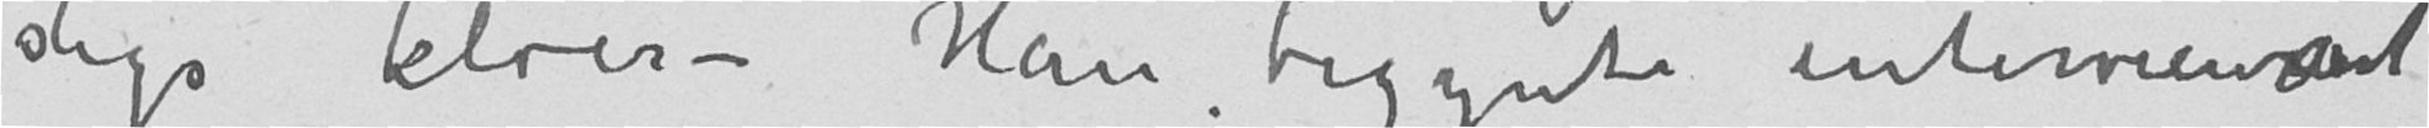slags kloer – Han begynte interviewet
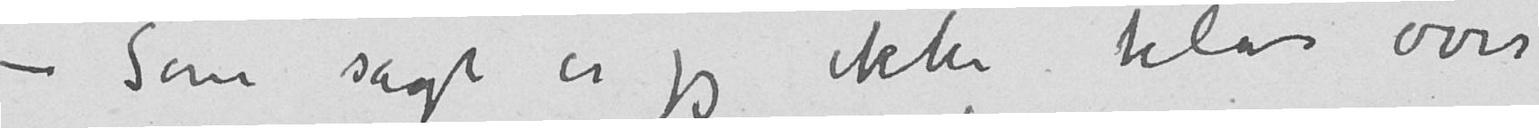– Som sagt er jeg ikke klar over
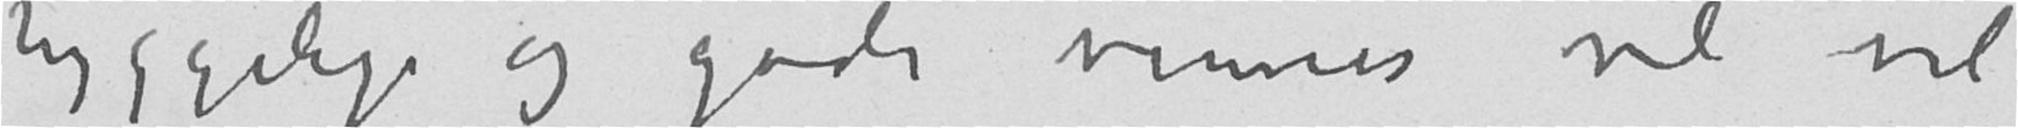hyggelige og gode venner vil vel
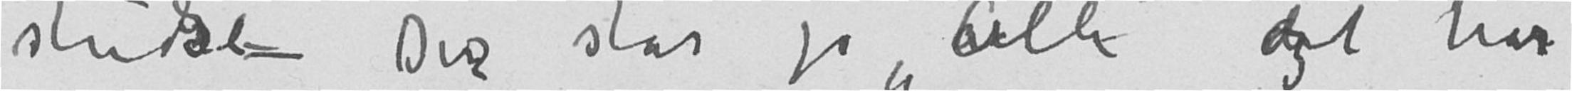studse – Der står jo «Alle» det har
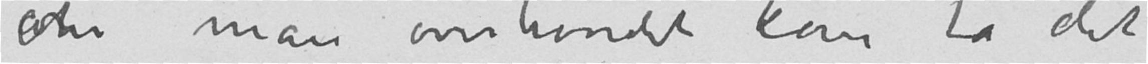om man overhovedet kan ta det
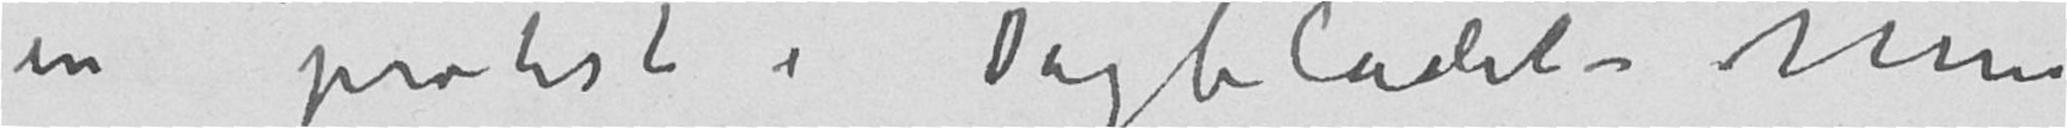en protest i Dagbladet – Men
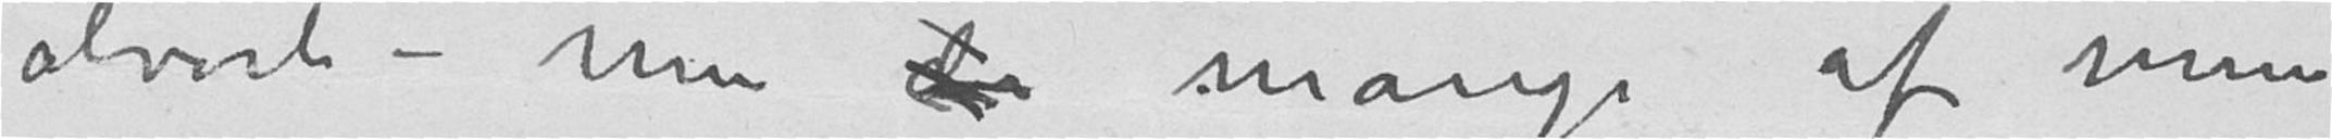alvorli – men da mange af mine
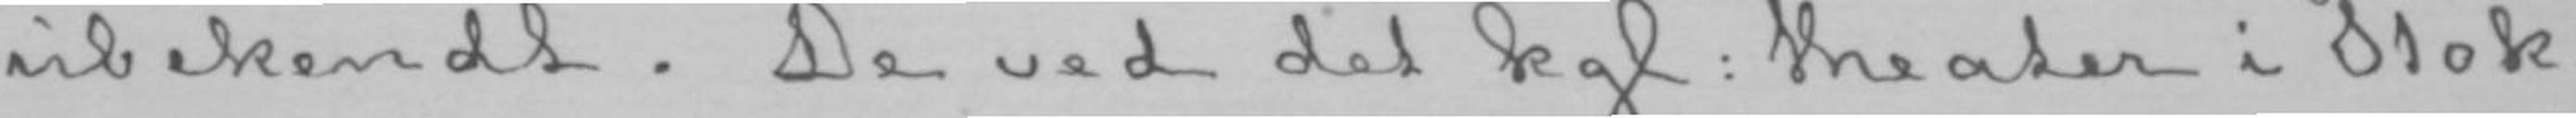ubekendt. De ved det kgl: theater i Stok-
